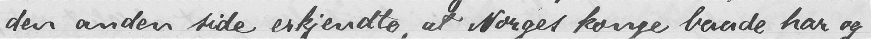den anden side erkjendte, at Norges konge baade har og
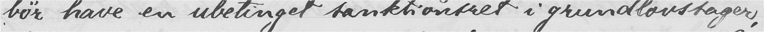bør have en ubetinget sanktionsret i grundlovssager,
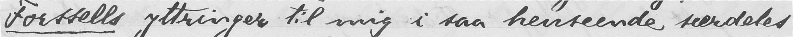Forssells yttringer til mig i saa henseende særdeles
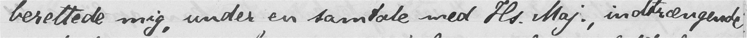berettede mig, under en samtale med Hs. Maj., indtrængende
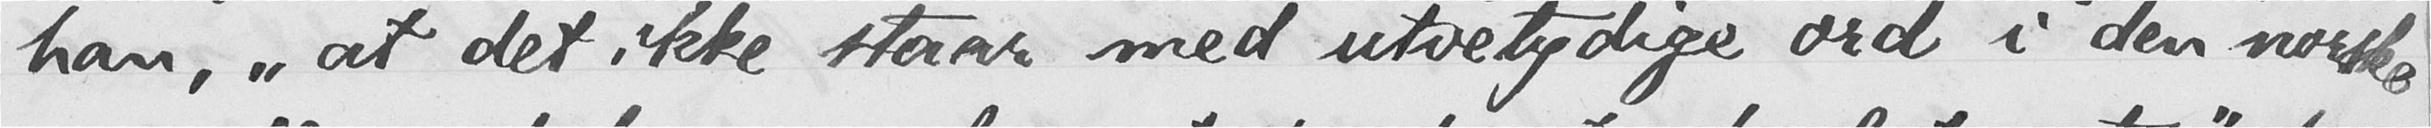han, "at det ikke staar med utvetydige ord i den norske
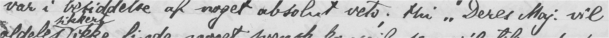var i besiddelse af noget absolut veto; thi "Deres Maj. vil
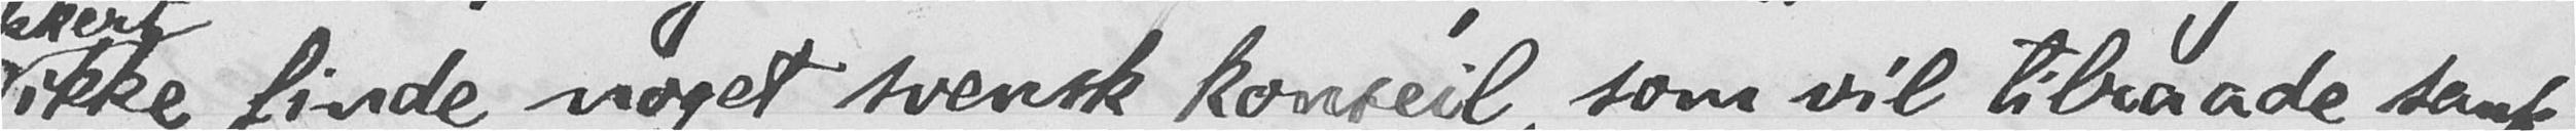ikke finde noget svensk konseil, som vil tilraade sank-
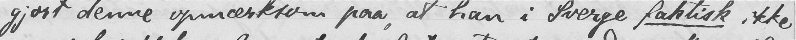gjort denne opmærksom paa, at han i Sverge faktisk ikke
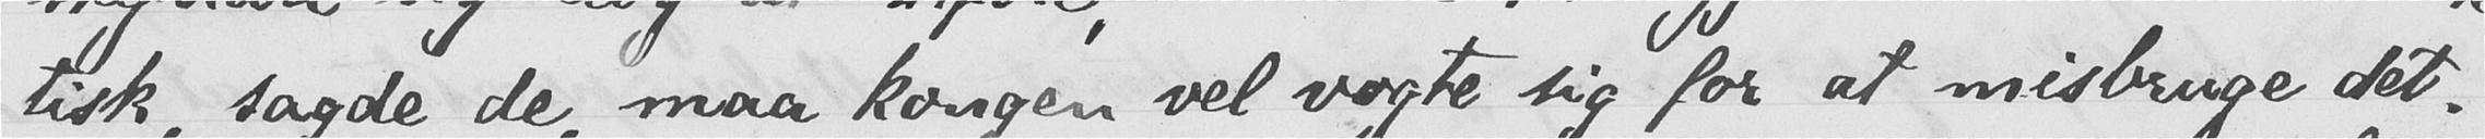tisk, sagde de, maa kongen vel vogte sig for at misbruge det.
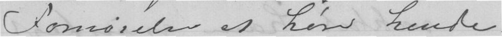Fornøielse at høre hende
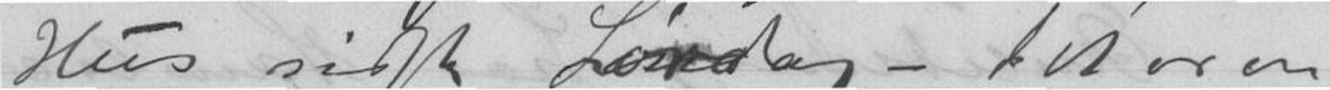Hus sidst Lørdag - Det er en
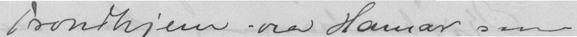Trondhjem via Hamar som
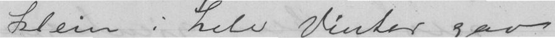klein i hele Vinter gav
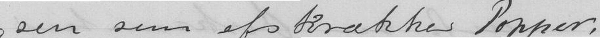sen som afskrekker Popper.
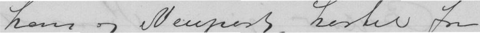ham og Neupert hertil fra
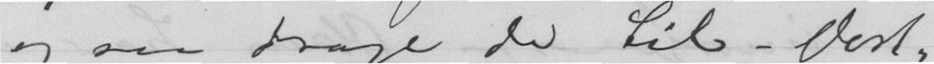og saa drager de til Vest-
